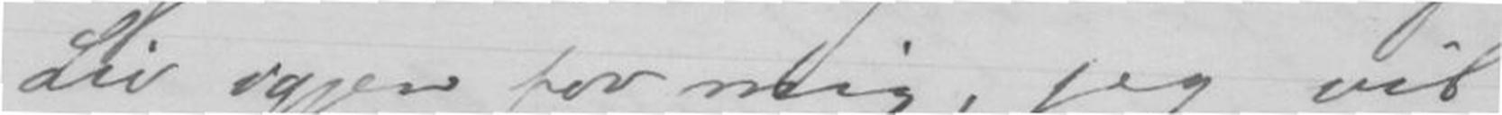Liv igjen for mig, jeg vil
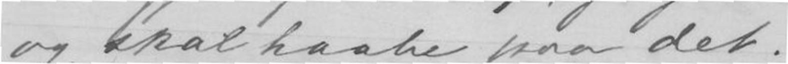og skal haabe paa det.
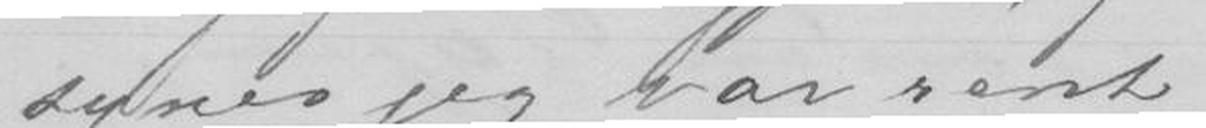synes jeg var rent
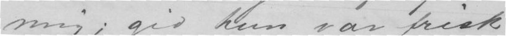mig; gid hun var frisk,
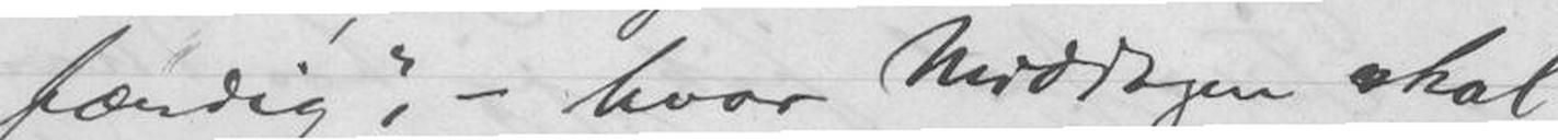færdig", - hvor Middagen skal
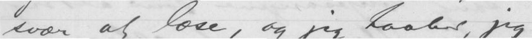svær at læse, og jeg haaber, jeg
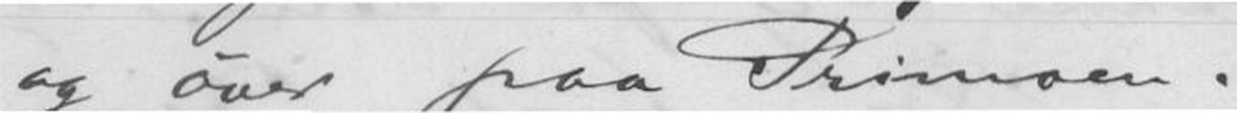og øver paa Pianoen.
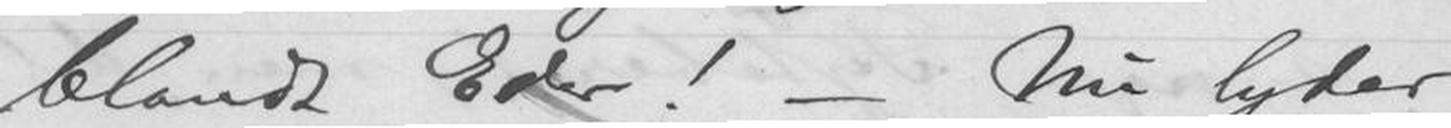blandt Eder! - Nu lyder
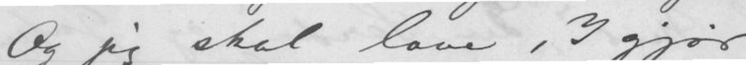Og jeg skal love, I gjør
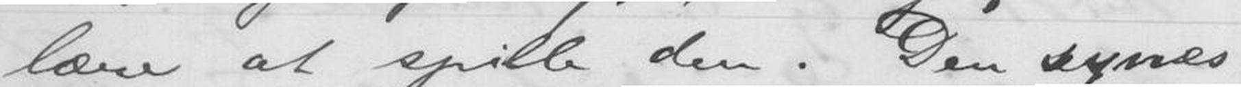lære at spille den. Den synes
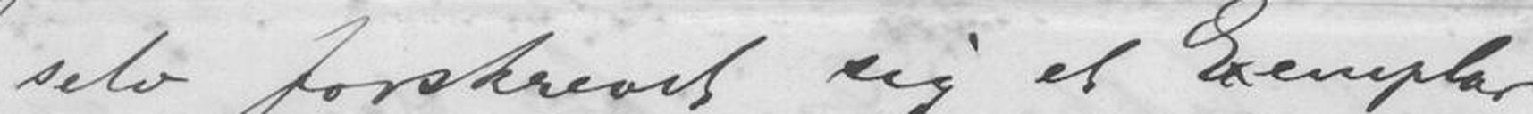selv forskrevet sig et Exemplar
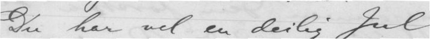Du har vel en deilig Jul
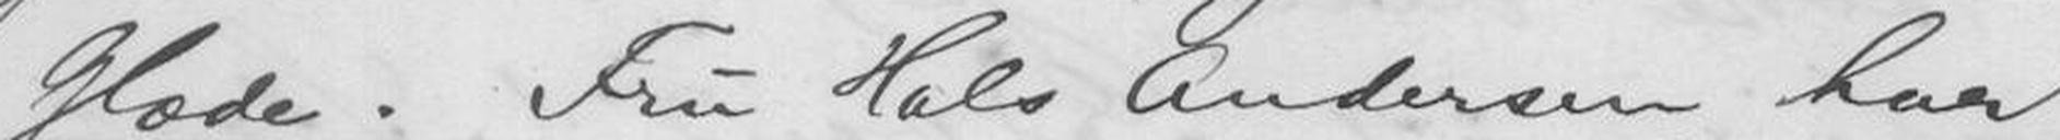Glæde. Fru Hals Andersen har
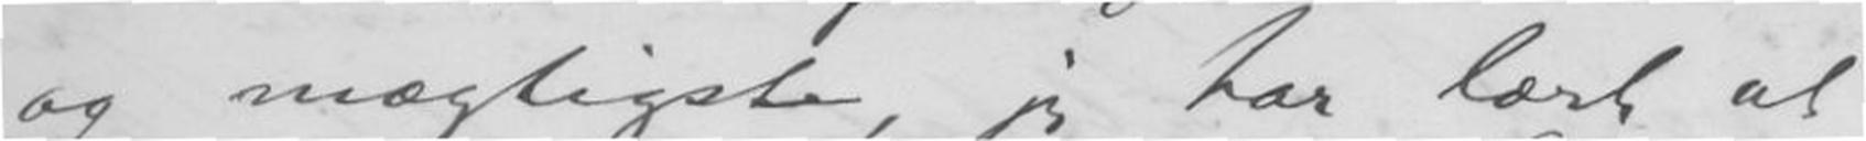og mægtigste, jeg har lært at
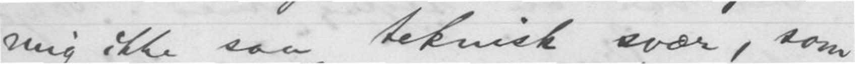mig ikke saa teknisk svær, som
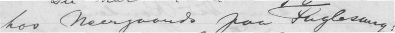hos Neergaards paa Fuglesang!
